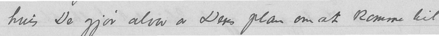hvis De gjør alvor av Deres plan om at komme til
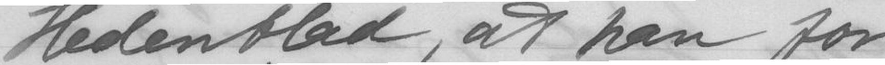Hedenblad, at han for
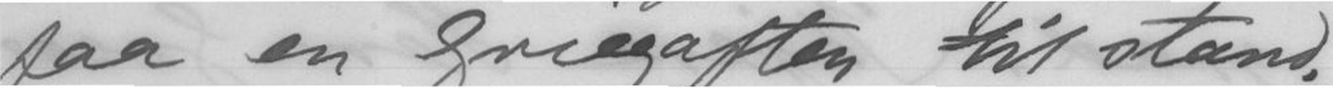paa en Griegaften til stand,
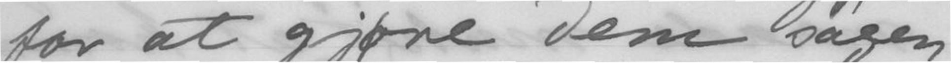for at gjøre Dem sagen
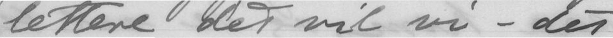lettere det vil vi - det
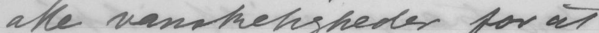alle vanskeligheder for at
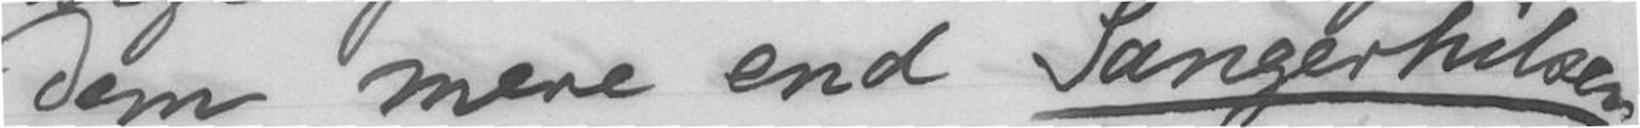Dem mere end Sangerhilsen
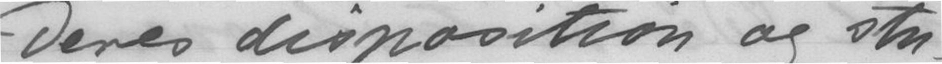Deres disposition og stu
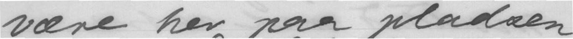være her paa pladsen
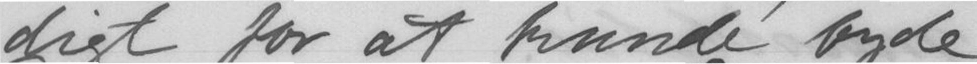digt for at kunde byde
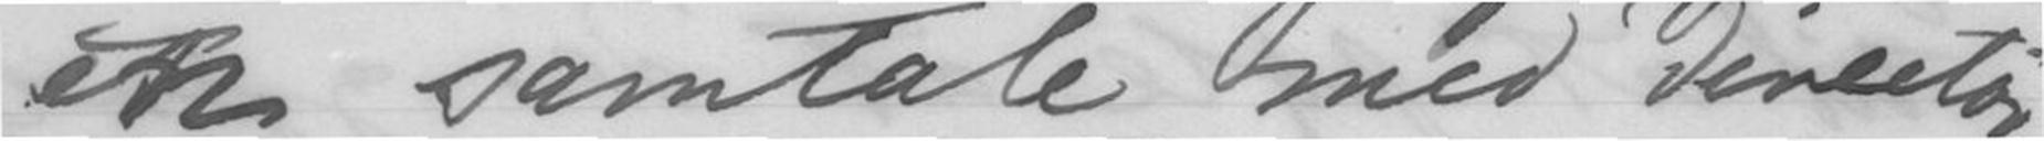en samtale med Directør
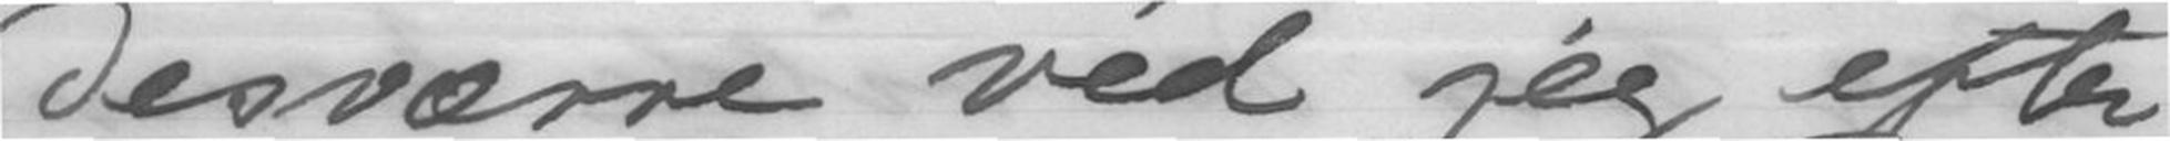Desværre ved jeg efter
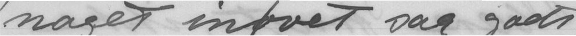noget inøvet saa godt
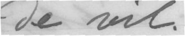De vil.
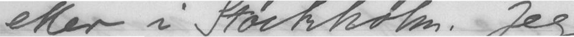eller i Stockholm. Jeg
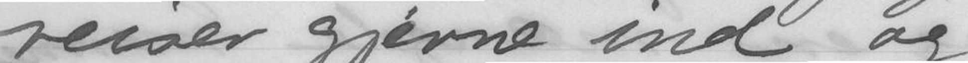reiser gjerne ind og
